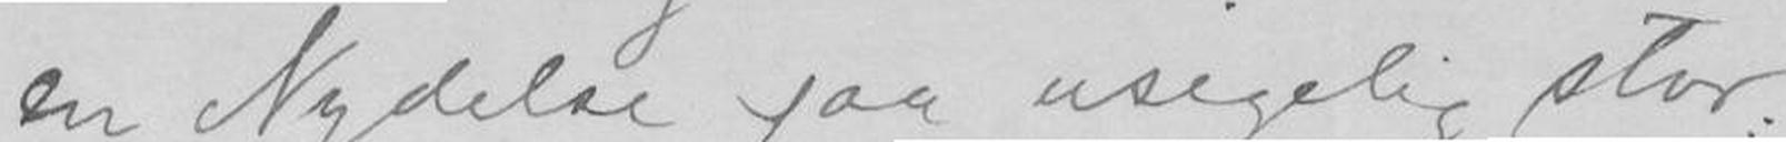en Nydelse saa usigelig stor
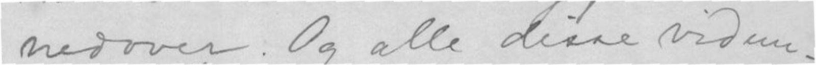nedover. Og alle disse vidun-
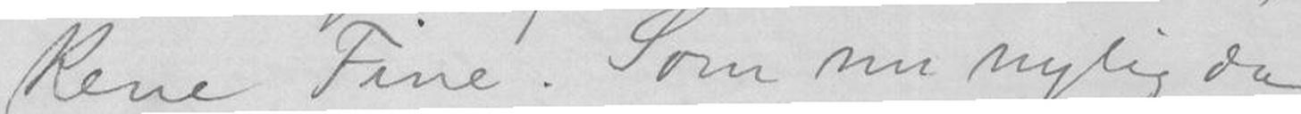Rene, Fine. Som nu nylig da
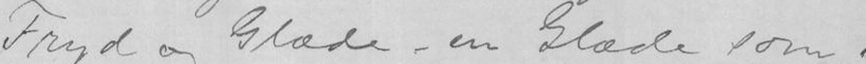Fryd og Glæde - en Glæde som
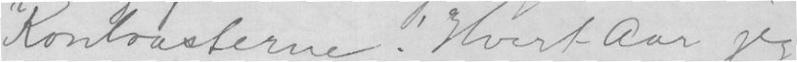Kontrasterne! Hvert Aar jeg
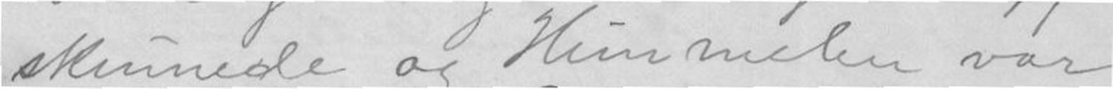skinnede og Himmelen var
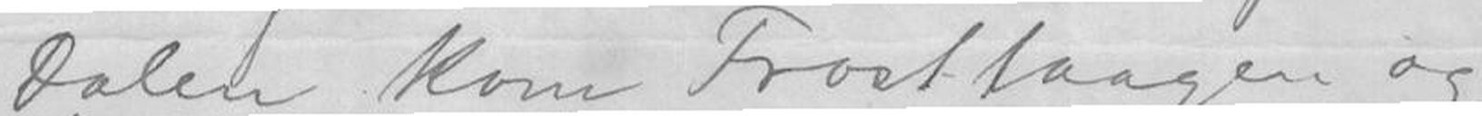Dalen kom Frosttaagen og
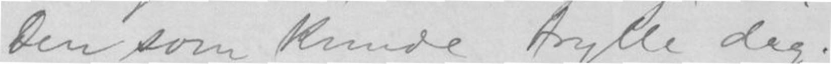Den som kunde trylle dig.
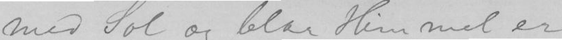med Sol og blaa Himmel er
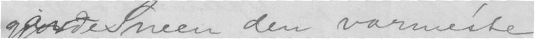gavgjorde Sneen den varmeste
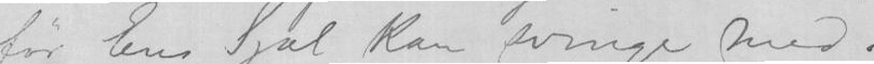før Ens Sjæl kan svinge med.
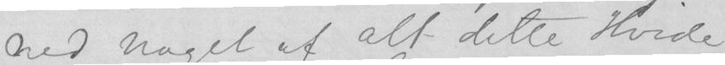ned Noget af alt dette Hvide
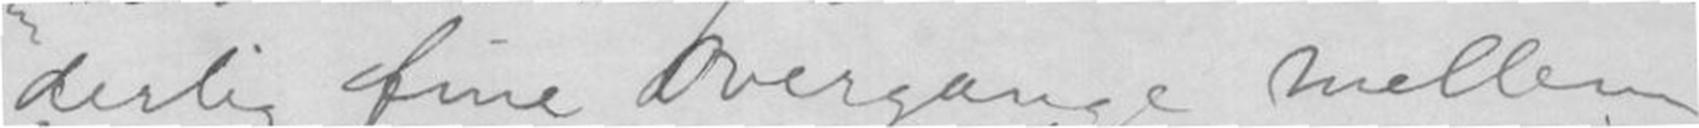derlig fine Overgange mellem
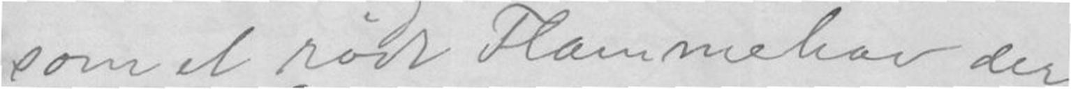som et rødt Flammehav, der
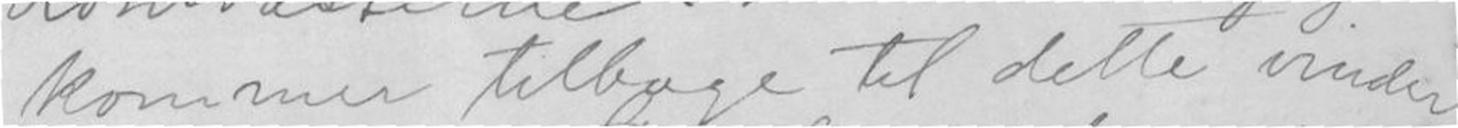kommer tilbage til dette vinder
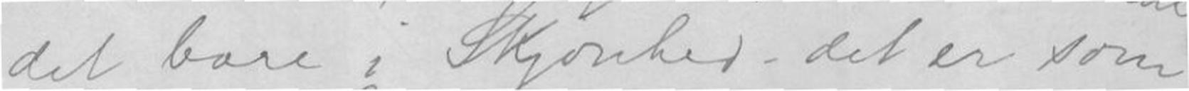det bare i Skjønhed det er som
